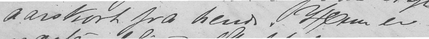aarskort fra hende. Hun er
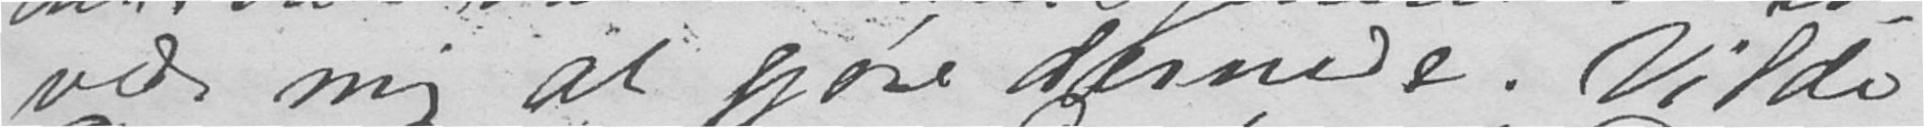vede mig at gjøre dernede. Vilde
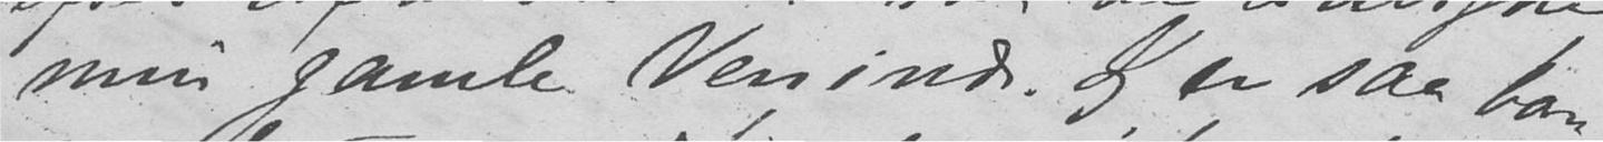min gamle Veninde. Jeg er saa ban-
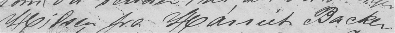Hilsen fra Harriet Backer.
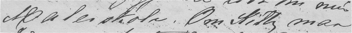Malerskole. Om Kitty maa
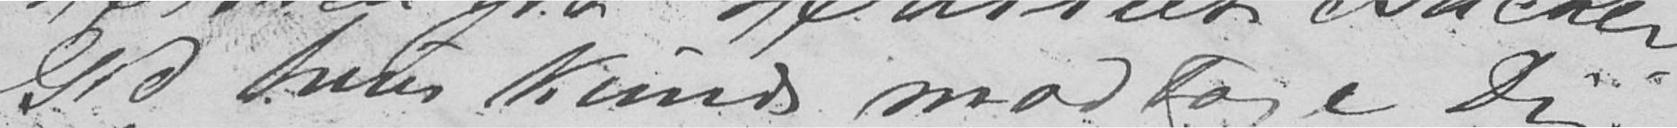Gid hun kunde modtage Dig.
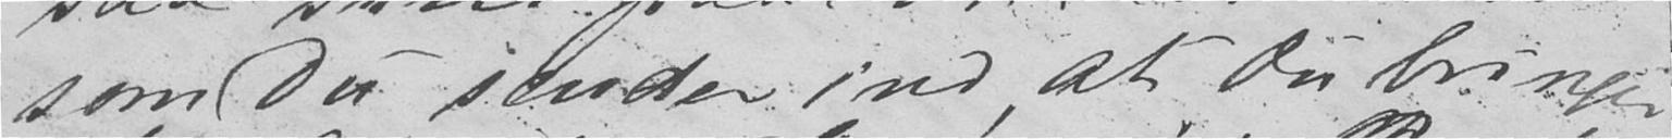som Du sender ind, at du bringer
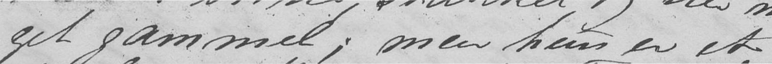get gammel; men hun er et
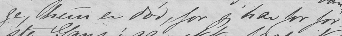ge, hun er død, for jeg har for før-
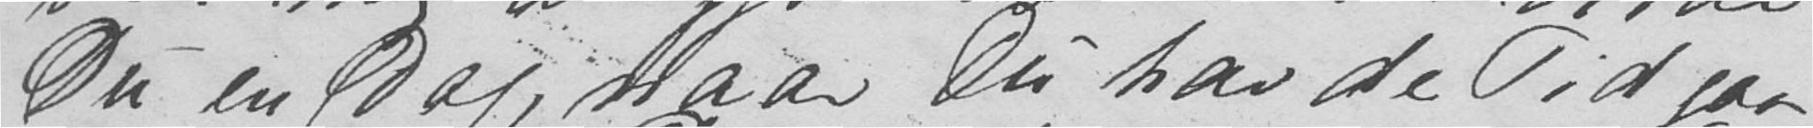Du en Dag, naar Du havde Tid gaa
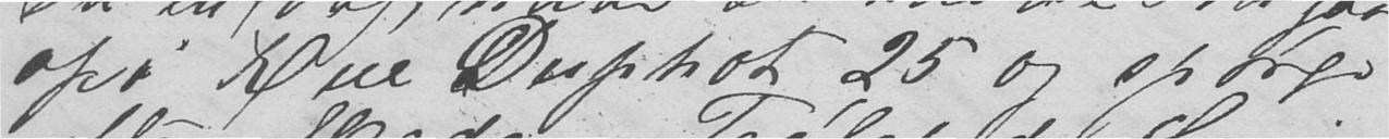op i Rue Duphot 25 og spørge
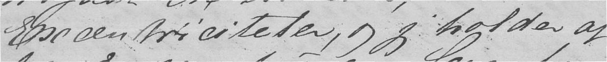Eccentriciteter, og jeg holder af
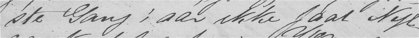ste Gang i aar ikke faat Nyt-
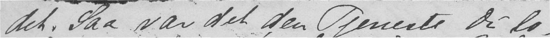det. Saa var det den Tjeneste du lo-
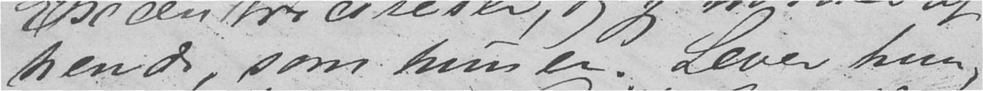hende, som hun er. Lever hun,
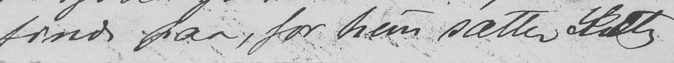finde paa, for hun sætter Kitty
